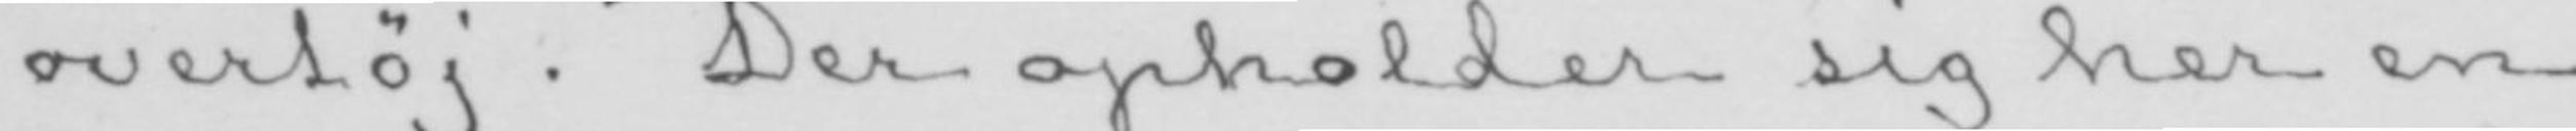overtøj. Der opholder sig her en
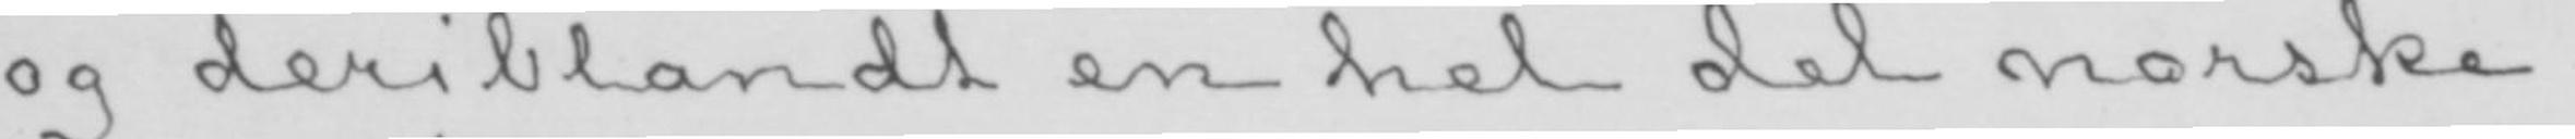og deriblandt en hel del norske.
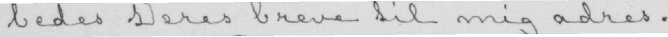bedes Deres breve til mig adres-
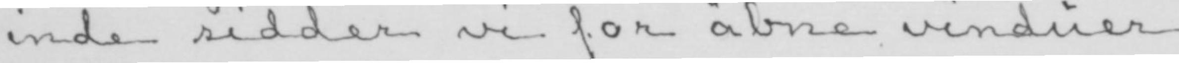inde sidder vi for åbne vinduer
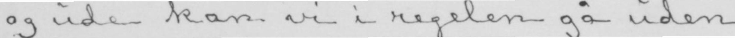og ude kan vi i regelen gå uden
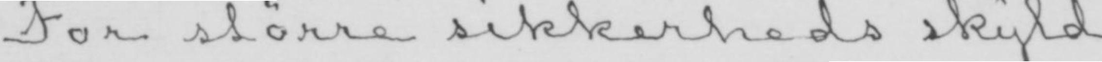For større sikkerheds skyld
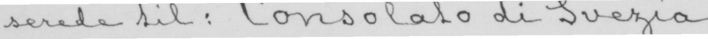serede til: Consolato di Svezia
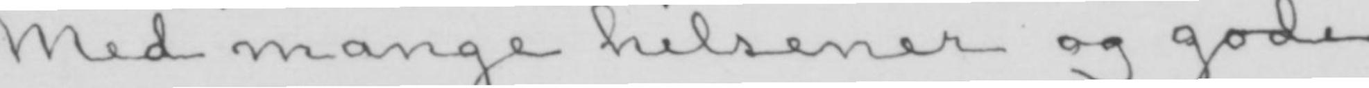Med mange hilsener og gode
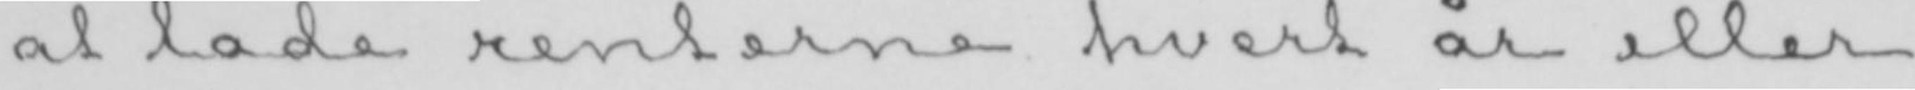at lade renterne hvert år eller
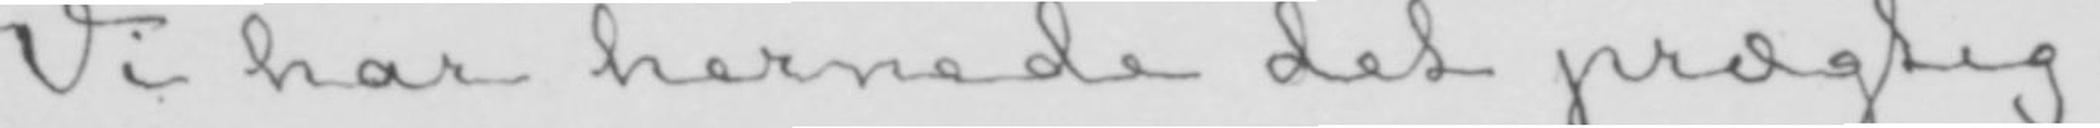Vi har hernede det prægtig-
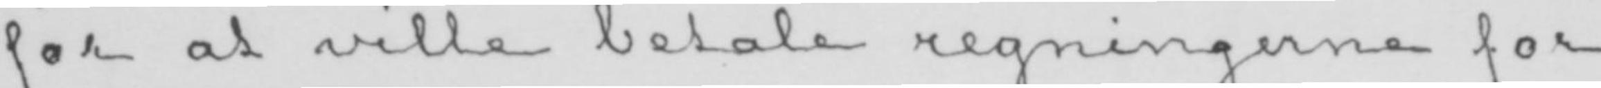for at ville betale regningerne for
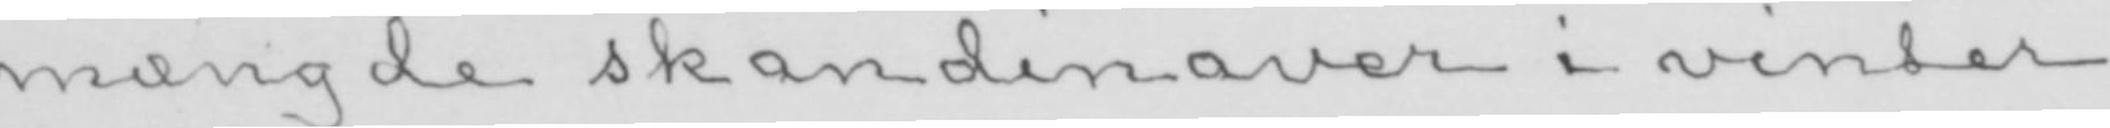mængde skandinaver i vinter
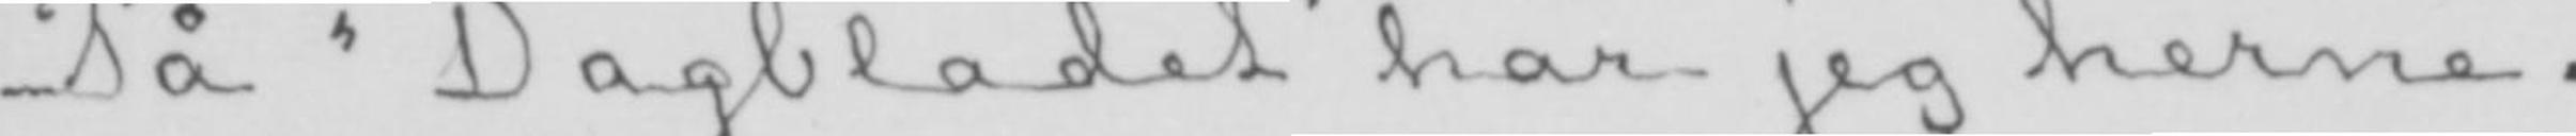På «Dagbladet» har jeg herne-
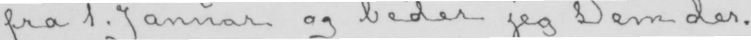fra 1. Januar og beder jeg Dem der-
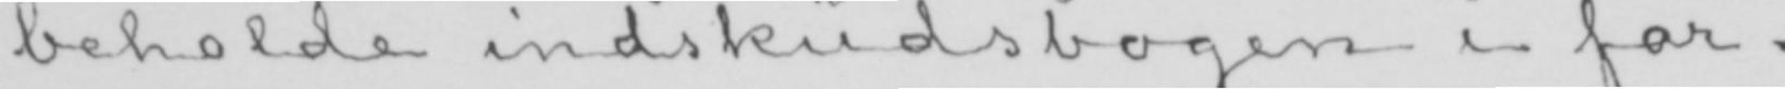beholde indskudsbogen i for-
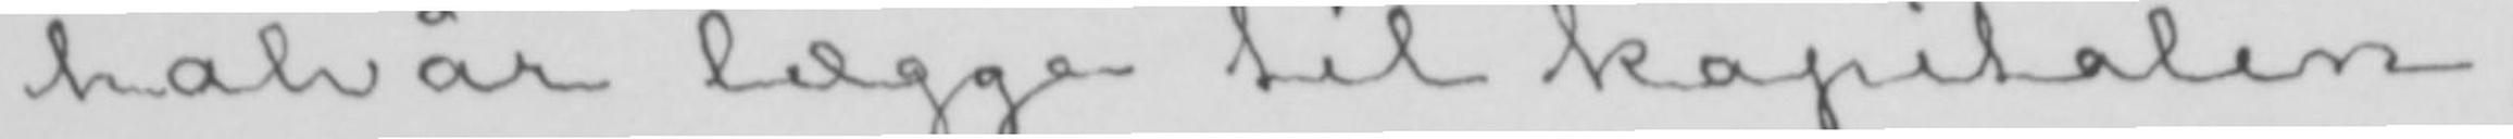halvår lægge til kapitalen,
